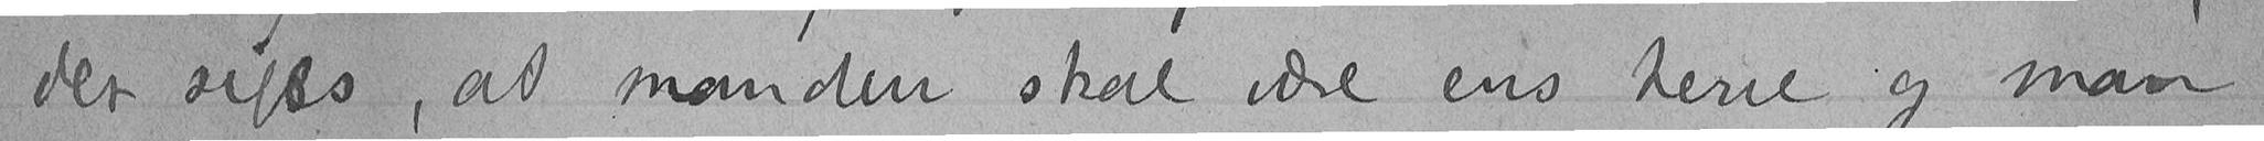der siges, at manden skal være ens herre og man
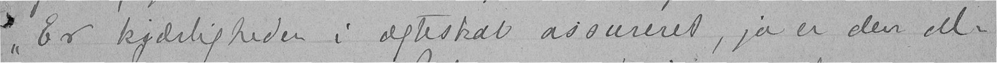"Er kjærligheden i ægteskab assureret, ja er den al-
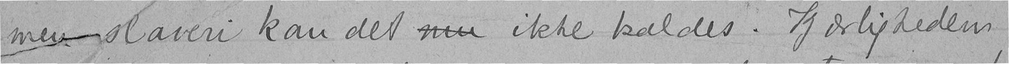men slaveri kan det mu ikke kaldes. Kjærligheden
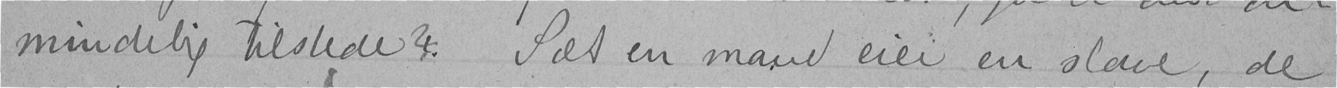mindelig tilstede? Sæt en mand eier en slave, de
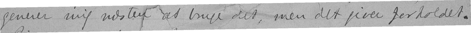generer mig næsten at bruge det, men det giver forholdet.
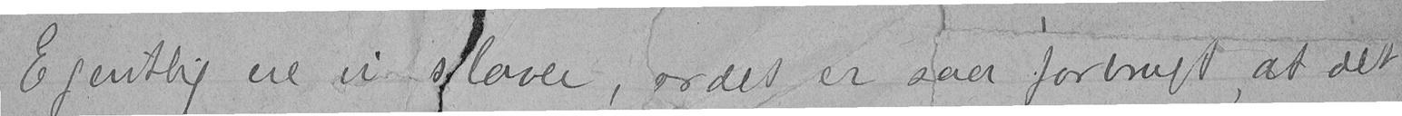Egentlig ere vi slaver, ordet er saa forbrugt, at det
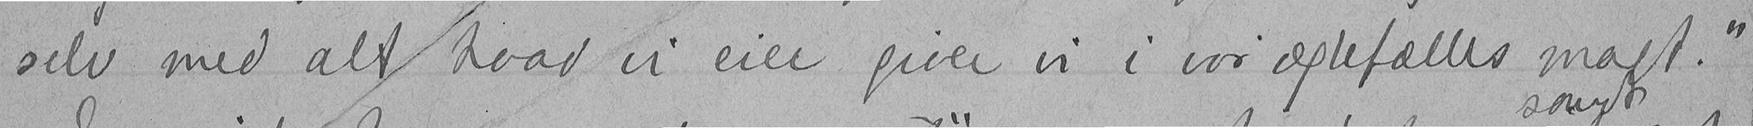selv med alt hvad vi eier giver vi i vor ægtefælles magt."
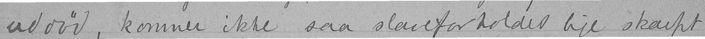uddød, kommer ikke saa slaveforholdet lige skarpt
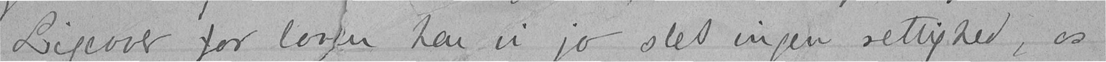Ligeover for loven har vi jo slet ingen rettighed, os
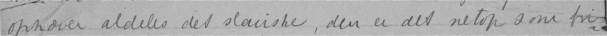ophæver aldeles det slaviske, den er det netop som fri-
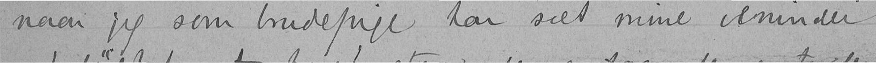naar jeg som brudepige har seet mine veninder
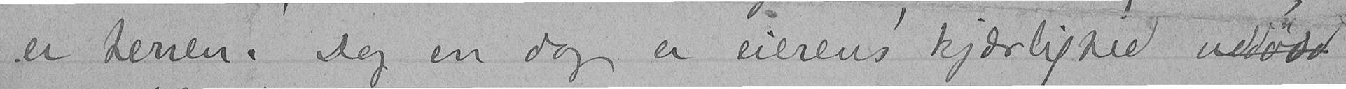er herren. Dog en dag er eierens kjærlighed uddøed
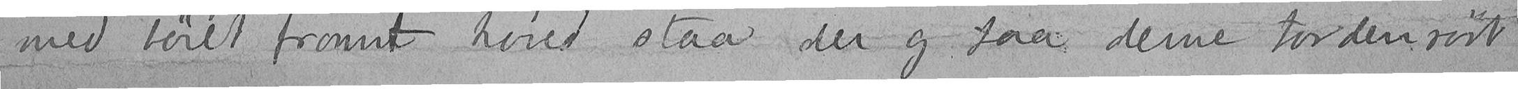med bøiet fromt hoved staa der og faa denne tordenrøst
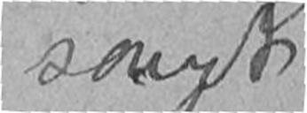sandt
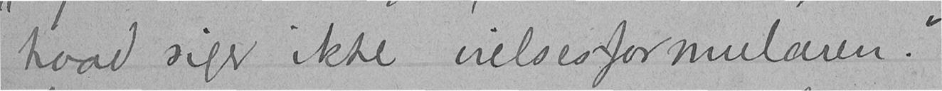hvad siger ikke vielsesformularen."
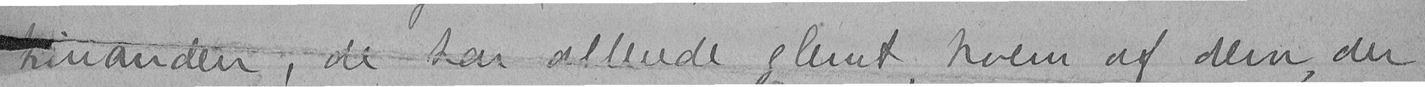hinanden, de har allerede glemt, hvem af dem, der
